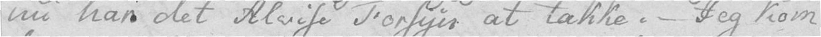nu har det Alvise Forsyn at takke. – Jeg kom
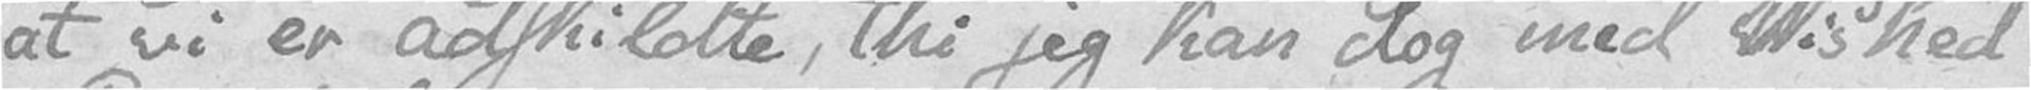at vi er adskildte, thi jeg kan dog med Vished
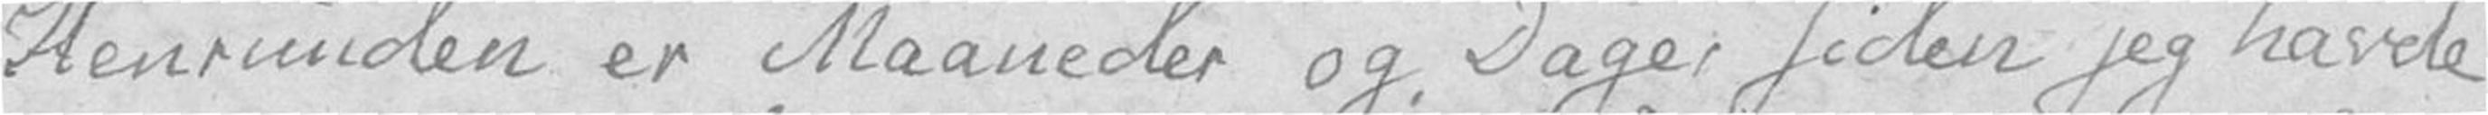Henrunden er Maaneder og Dager, siden jeg havde
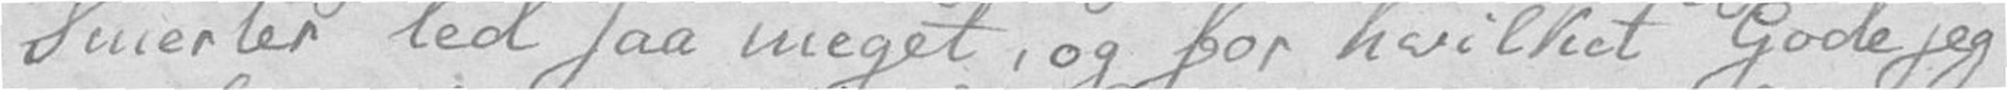Smerter led saa meget, og for hvilket Gode jeg
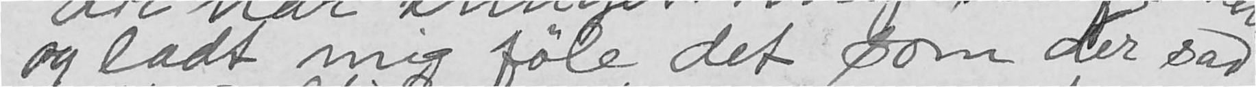og ladt mig føle det som der sad
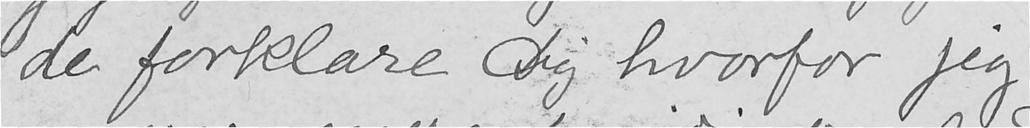de forklare Dig hvorfor jeg
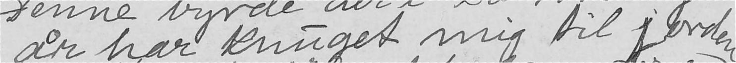år har knuget mig til jorden
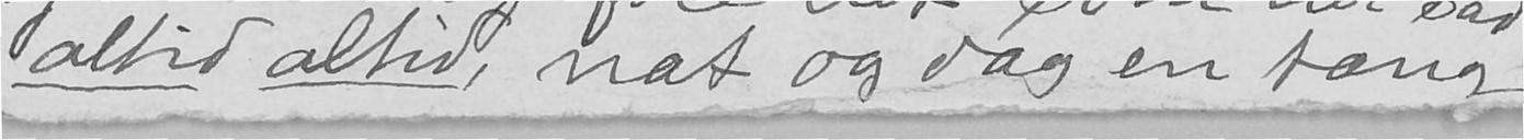altid altid, nat og dag en tang
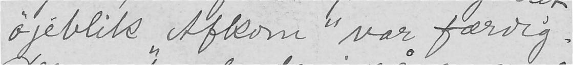øjeblik "Afkom" var færdig.
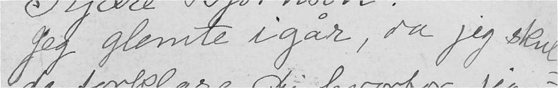Jeg glemte igår, da jeg skul-
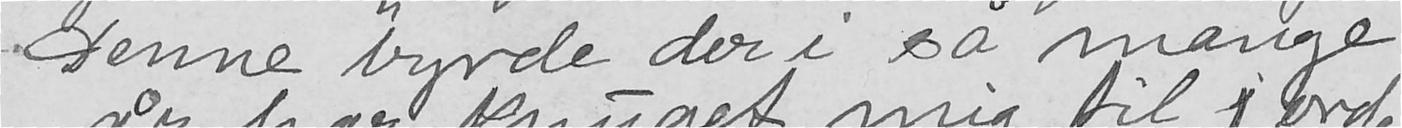Denne byrde der i så mange
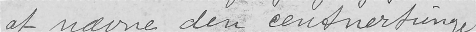at nævne den centnertunge
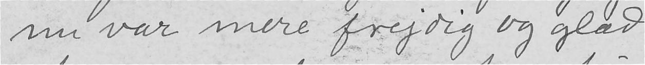nu var mere frejdig og glad
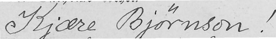Kjære Bjørnson!
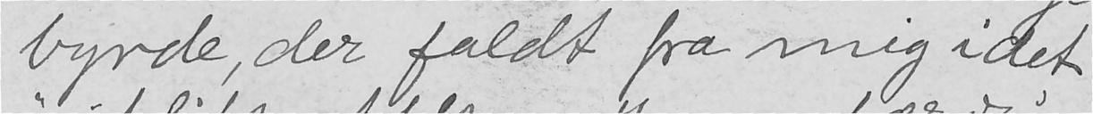byrde, der faldt fra mig idet
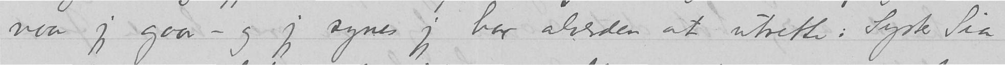naar jeg gaar – og jeg synes jeg har alverden at utrette: Syster Pia
